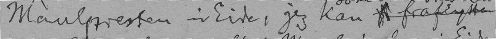Maulpresten i Eide, jeg kan f fraflytte
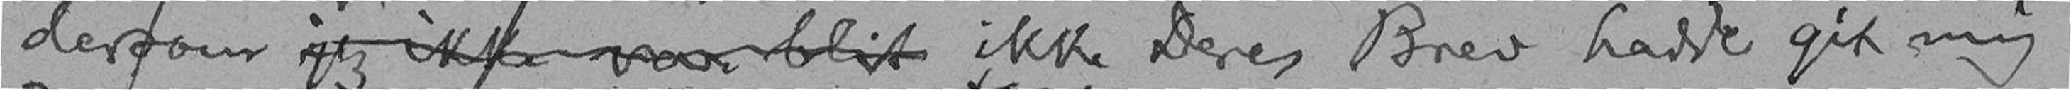dersom jeg ikke var blit ikke Deres Brev hadde git mig
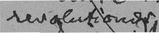revolutionær
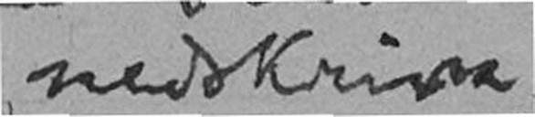nedskrive
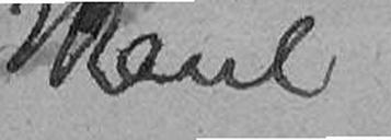Maul
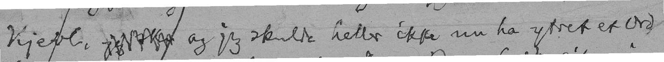Kjevl, jeg skr og jeg skulde heller ikke nu ha ytret et Ord
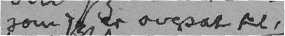som jeg er ovesat til,
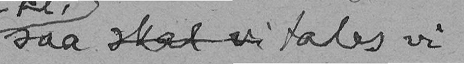saa skal vi tales vi
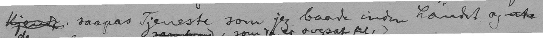kjendt saapas Tjeneste som jeg baade inden Landet og ute
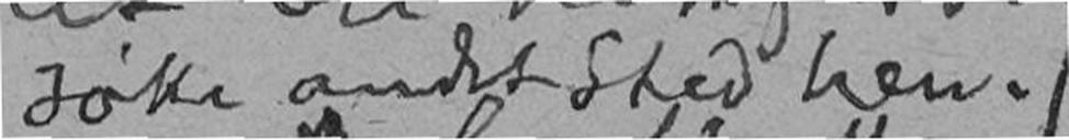søke andet Sted hen.
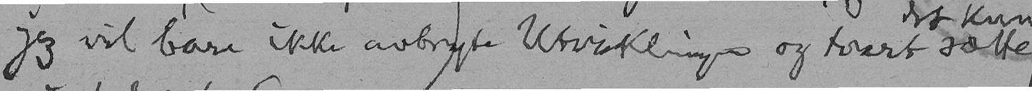jeg vil bare ikke avbryte Utviklingen og tvært sætte
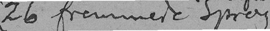26 fremmede Sprog
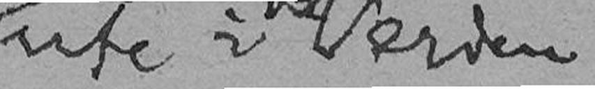ute i Verden
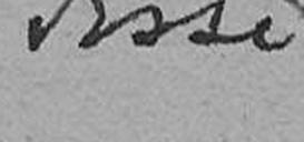disse
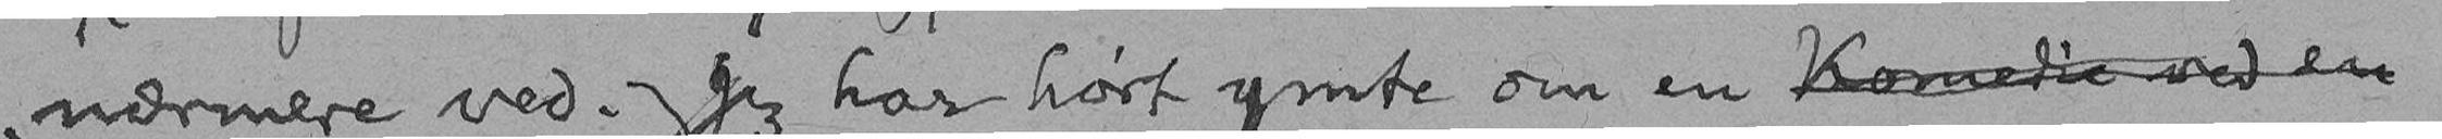nærmere ved. Jeg har hørt ymte om en Komedie ved en
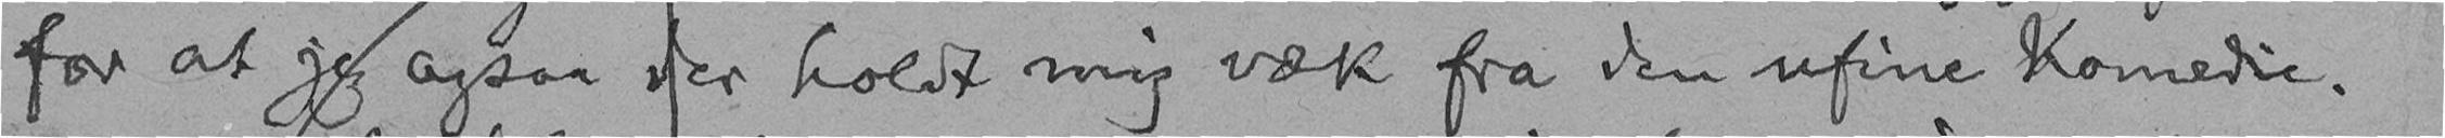for at jeg ogsaa der holdt mig væk fra den ufine Komedie.
